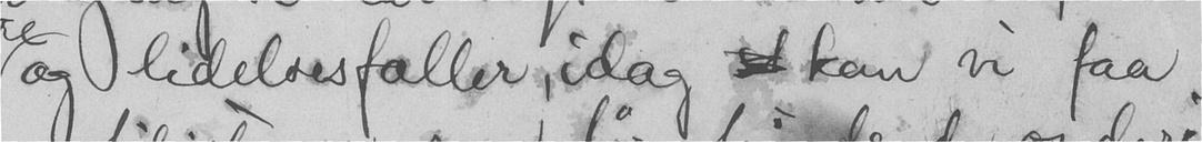og lidelsesfaller, idag  kan vi faa
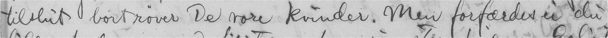tilslut bortrøver De vore kvinder. Men forfærdes ei du
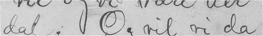dal. Og vil vi da
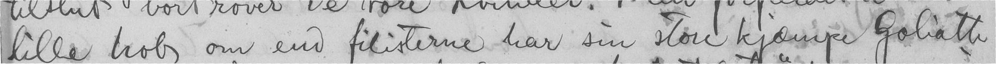lille hob om end filisterne har sin store kjæmpe Goliatte
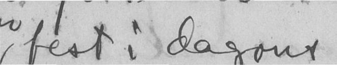fest i dagons
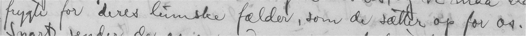frygte for deres lumske fælder, som de sætter op for os.
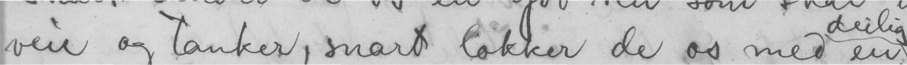veie og tanker, snart lokker de os med en
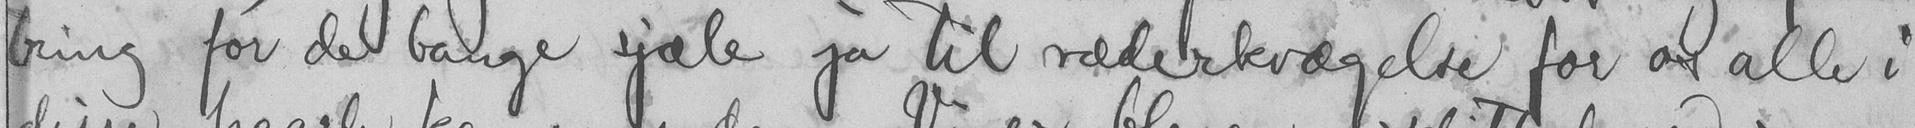tring for de bange sjæle ja til væderkvægelse for os alle i
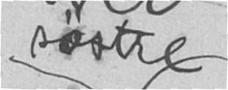søstre
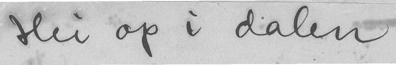Hei op i dalen
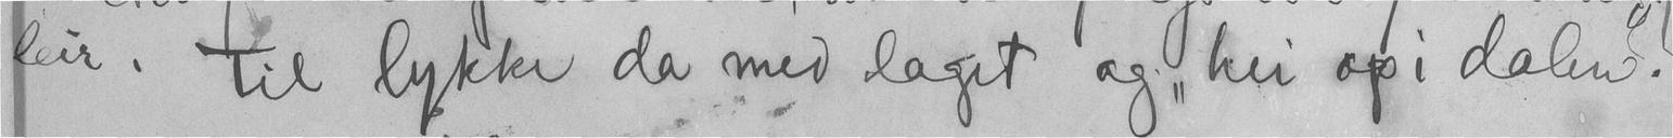leir. Til lykke da med laget og "hei op i dalen".
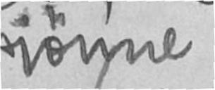sjønne
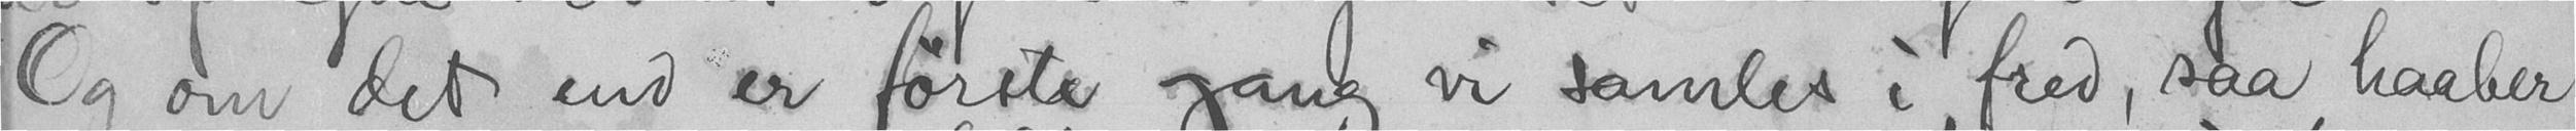Og om det end er første gang vi samles i fred, saa haaber
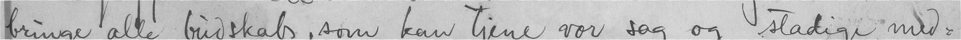bringe alle budskab, som kan tjene vor sag og stadige med-
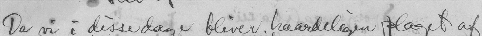Da vi i disse dage bliver haardeligen plaget af
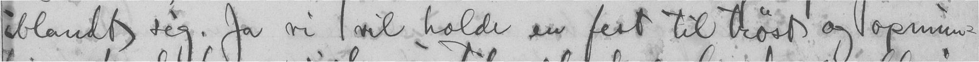iblandt sig. Ja vi vil holde en fest til trøst og opmun-
